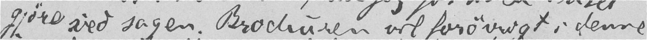gjøre ved sagen. Brochuren vil forøvrigt i denne
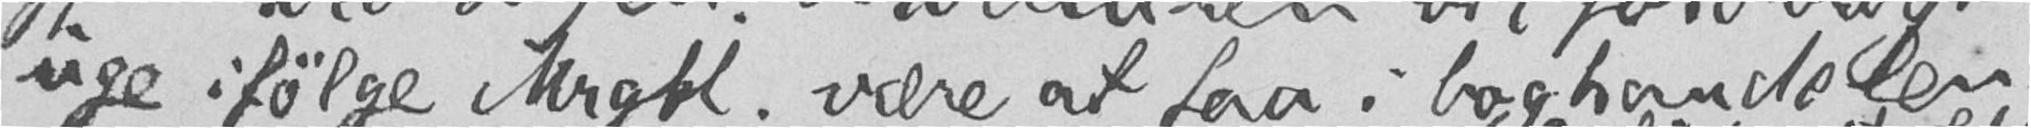uge ifølge Mrgbl. være at faa i boghandelen.
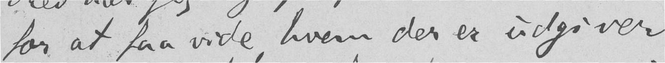for at faa vide, hvem der er udgiver
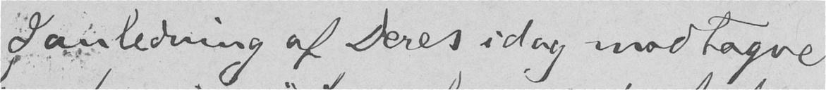I anledning af Deres i dag modtagne
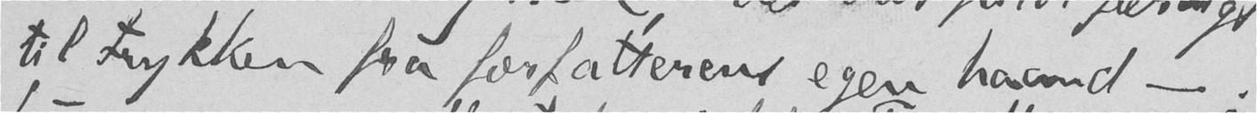til trykken fra forfatterens egen haand - .
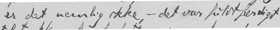er det nemlig ikke, - det var fuldt færdigt
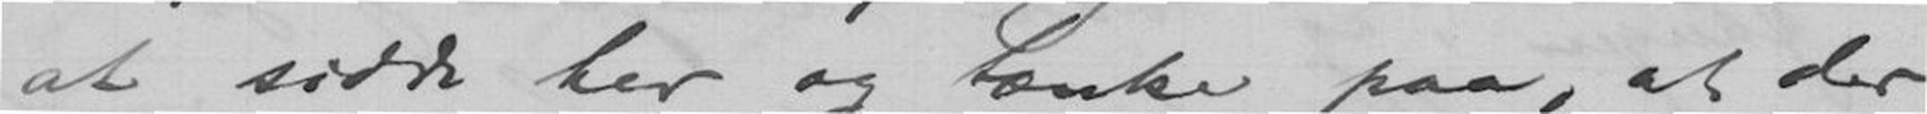at sidde her og tænke paa, at der
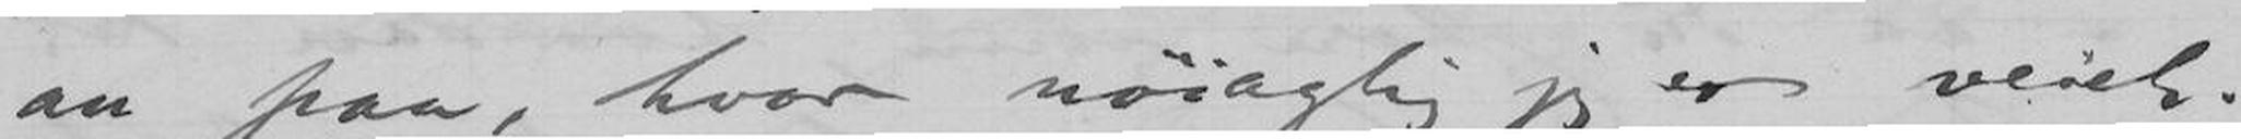an paa, hvor nøiagtig jeg er veiet.
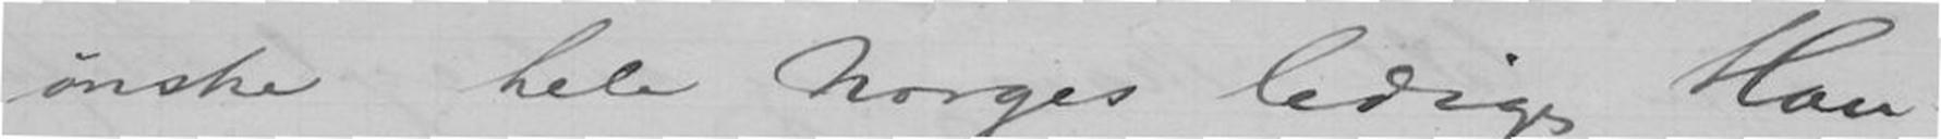ønske hele Norges ledige Han-
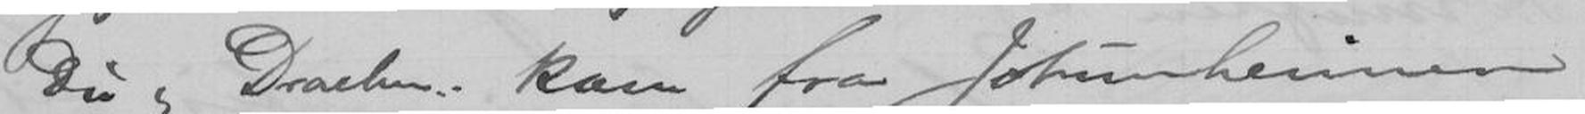Du og Drachm. kom fra Jotunheimen -
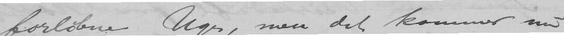forløbne Uge, men det kommer nu
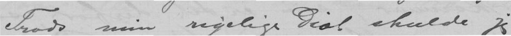Trods min rigelige Diæt skulde jeg
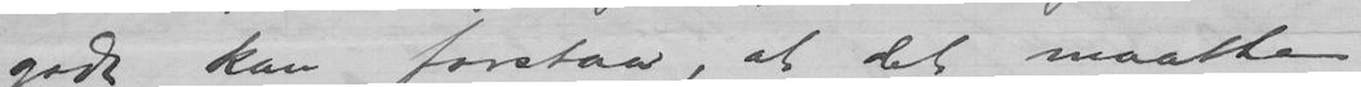godt kan forstaa, at det maatte
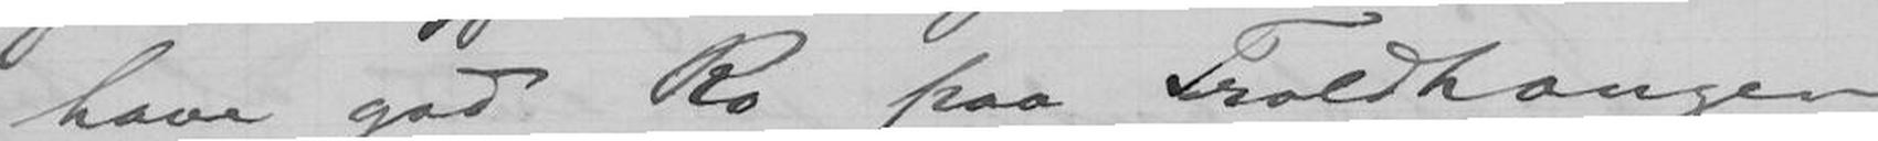have god Ro paa Troldhougen
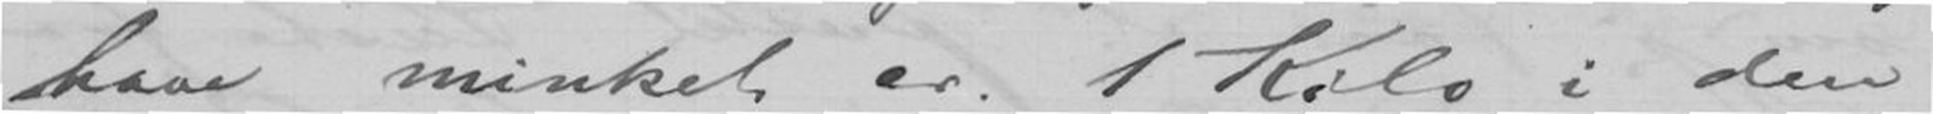have minket ca. 1 Kilo i den
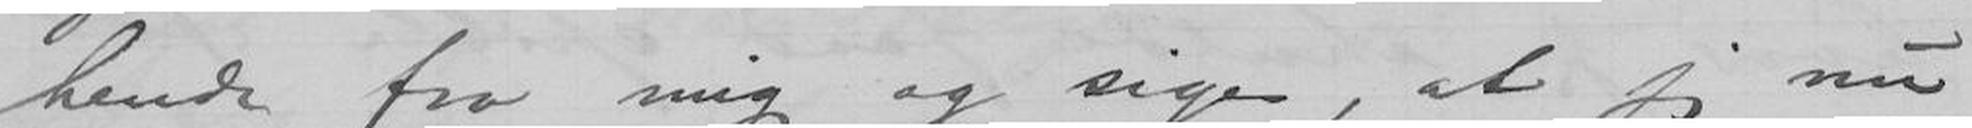hende fra mig og sige, at jeg nu
